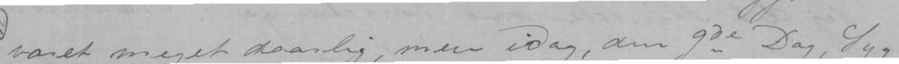varet meget daarlig, men idag, den 9de Dag, Syg-
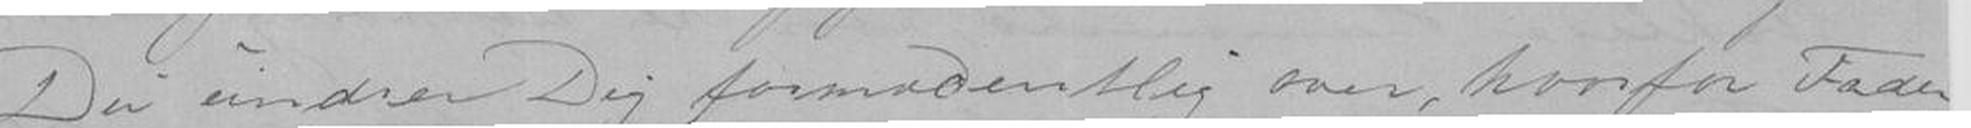Du undrer Dig formodentlig over, hvorfor Fader
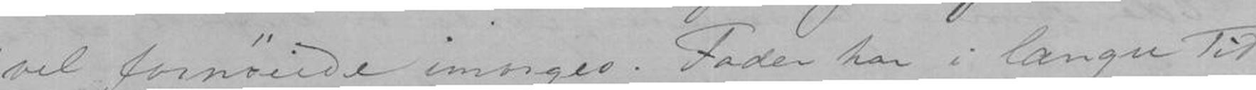vel fornøiede imorges. Fader har i længre Tid
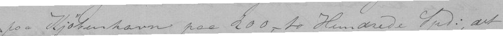paa Kjøbenhavn paa 200,- to Hundrede Spd:, det
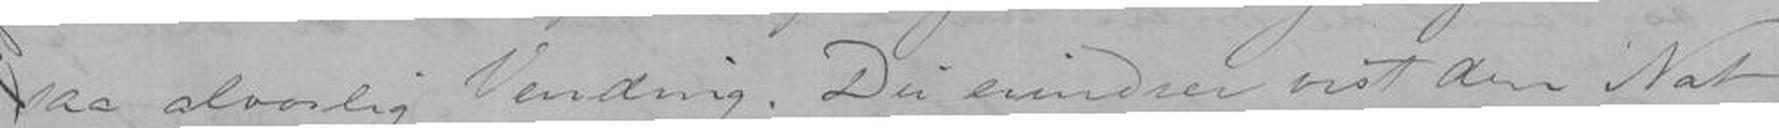saa alvorlig Vending. Du erindrer vist den Nat,
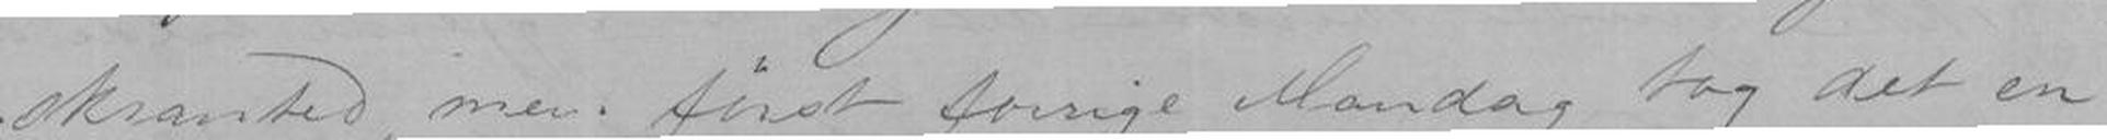skranted, men først forrige Mandag tog det en
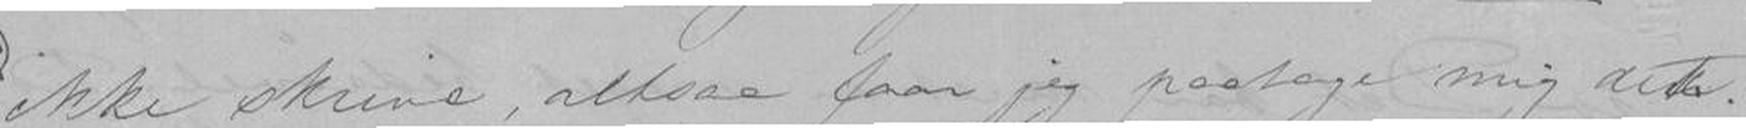ikke skrive, altsaa faar jeg paatage mig det.
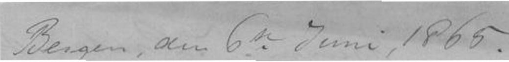Bergen, den 6te Juni, 1865.
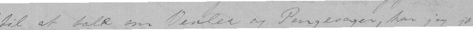til at tale om Vexler og Pengesager, har jeg jo
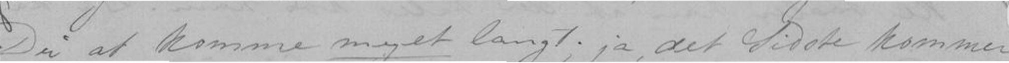Du at komme meget langt; ja, det Sidste kommer
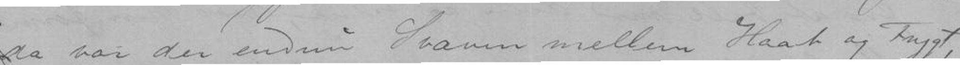da var der endnu Svæven mellem Haab og Frygt,
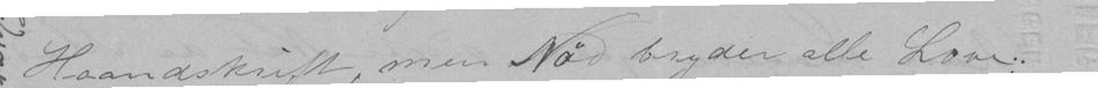Haandskrift, men Nød bryder alle Love,
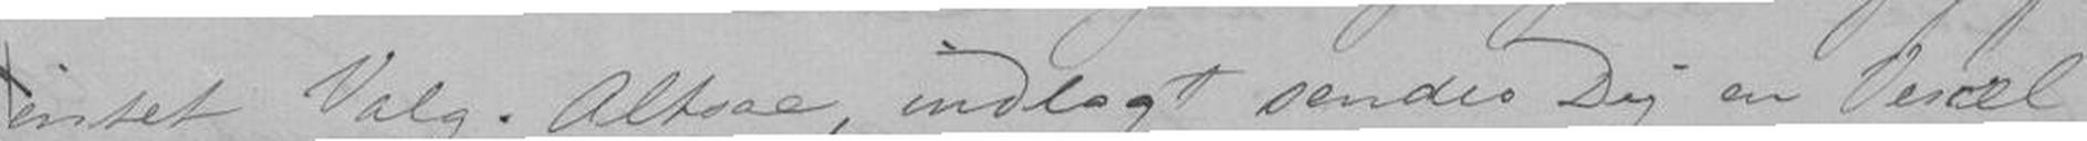intet Valg. Altsaa, indlagt sendes Dig en Vexel
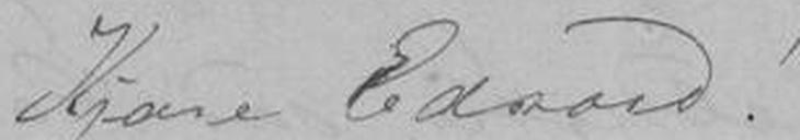Kjære Edvard!
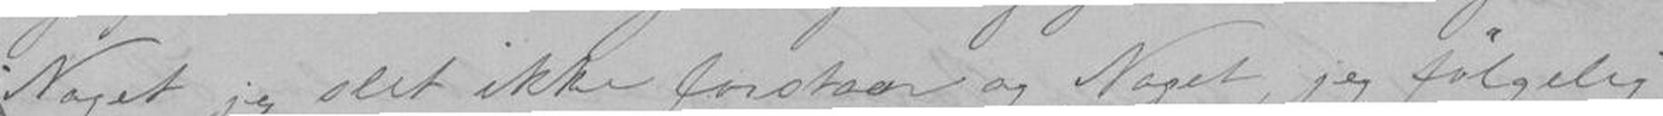Noget jeg slet ikke forstaar og Noget jeg følgelig
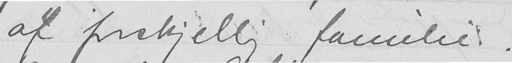af forskjellig familie.
